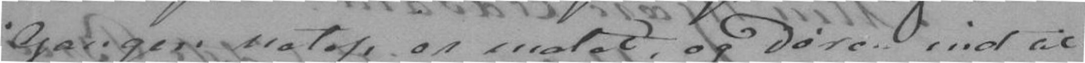Gangen netop er malet, og Døren ind til
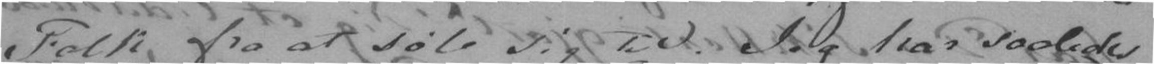Folk fra at søle sig til. Jeg har saaledes
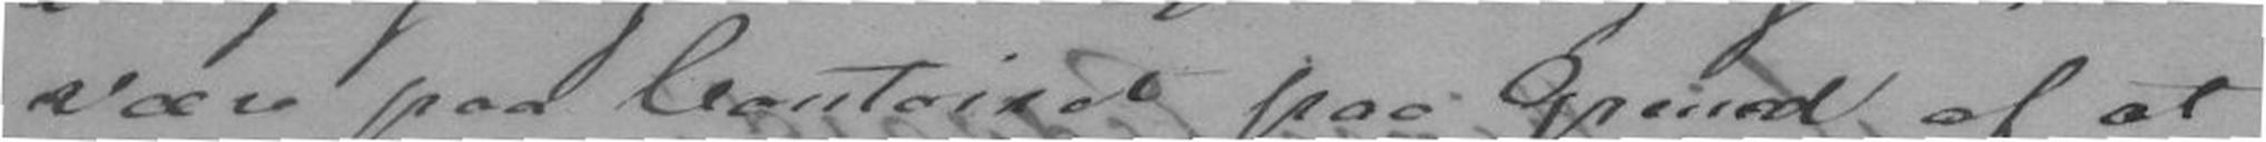være paa Contoiret paa Grund af at
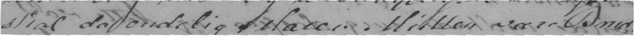skal da endelig Maren Müller være Brud.
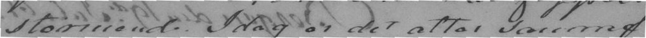stormende. Idag er det atter samme
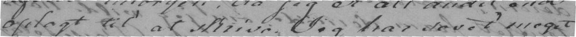oplagt til at skrive. Jeg har sovet meget
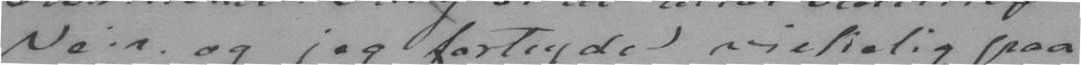Veir, og jeg fortryder virkelig paa
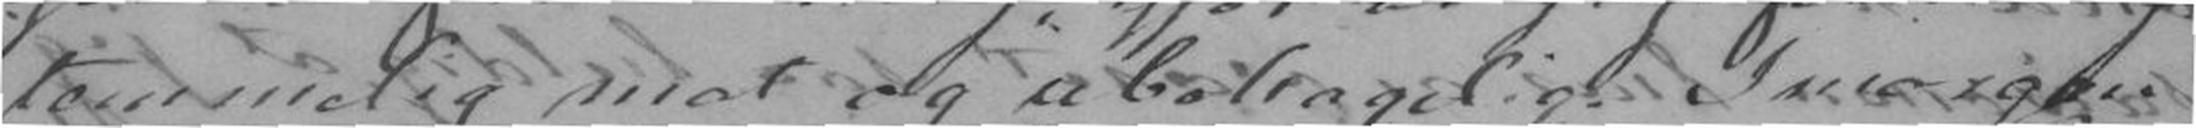temmelig mat og ubehagelig. Imorgen
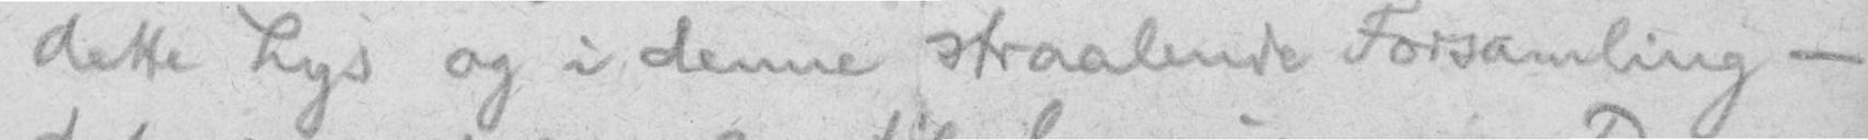dette Lys og i denne straalende Forsamling -
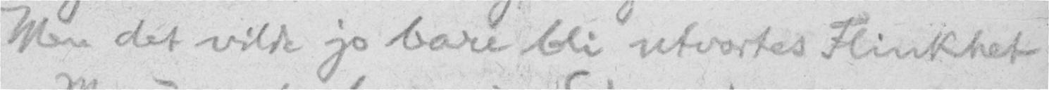Men det vilde jo bare bli utvortes Flinkhet
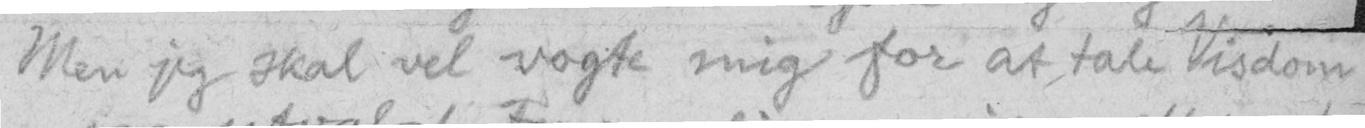Men jeg skal vel vogte mig for at tale Visdom
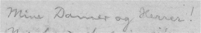Mine Damer og Herrer!
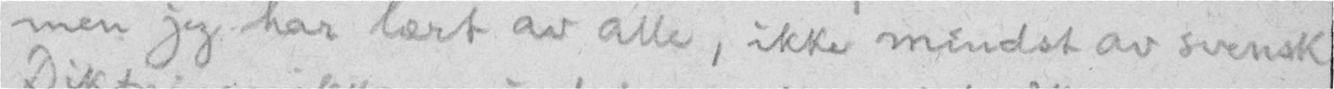men jeg har lært av alle, ikke mindst av svensk
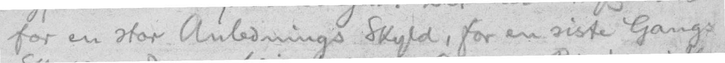for en stor Anlednings Skyld, for en siste Gangs
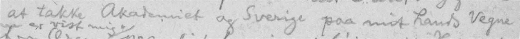at takke Akademiet og Sverige paa mit Lands Vegne
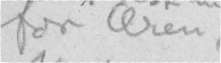for Æren
